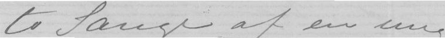to Sange af en ung
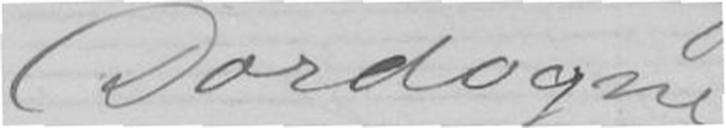Dordogne
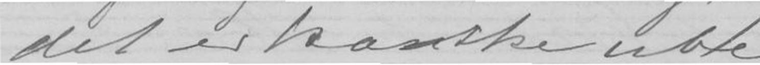det er kanske ube-
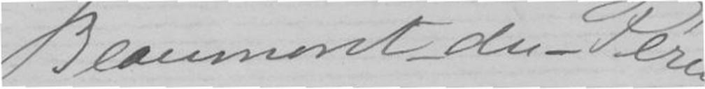Beaumont-du-Périgord
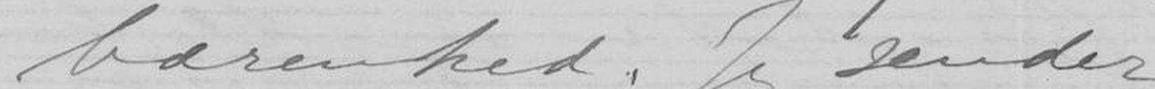bærenhed. Jeg sender
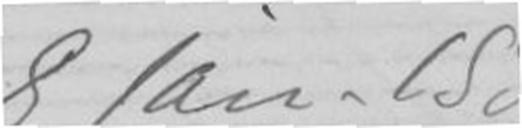8 jan. 1889
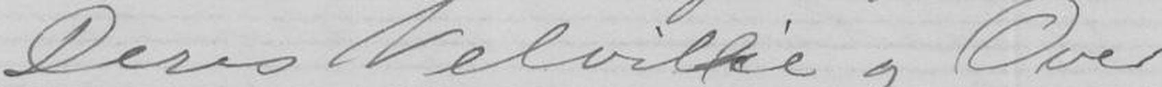Deres Velvilie og Over-
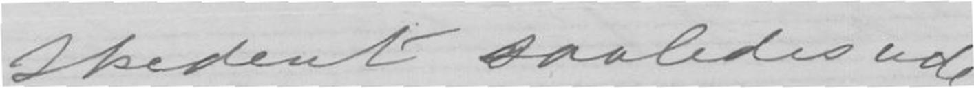skedent saaledes uden
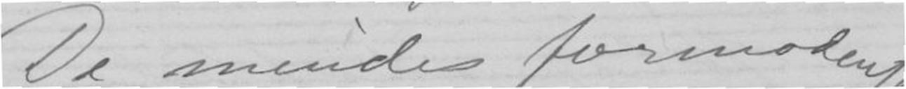De mindes formodentlig
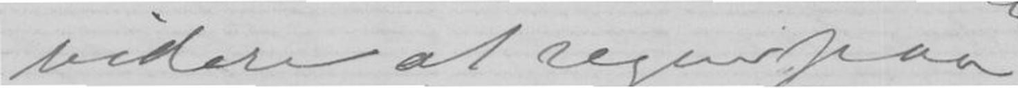videre at regne paa
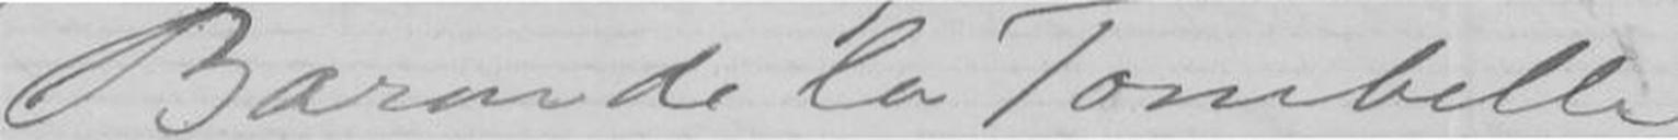Baroide la Tombelle
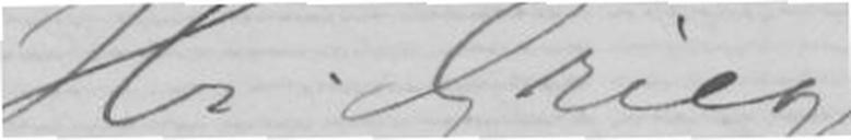Hr Grieg
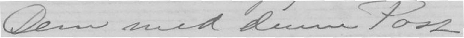Dem med denne Post
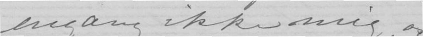engang ikke mig, og
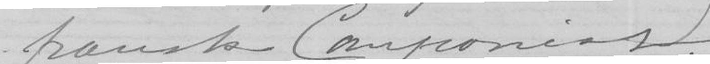fransk Componist,
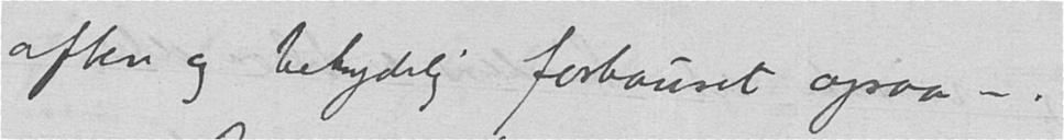aften og betydelig forbauset ogsaa –.
